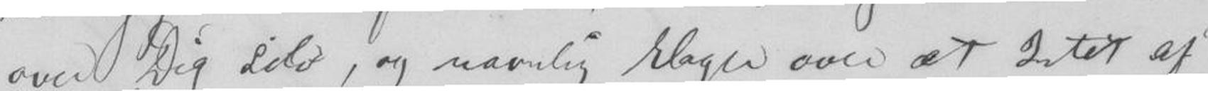over Dig selv, og navnlig klager over at Intet af
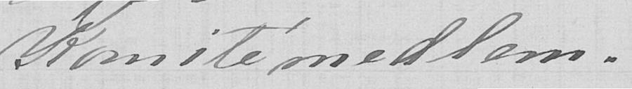Komitemedlem.
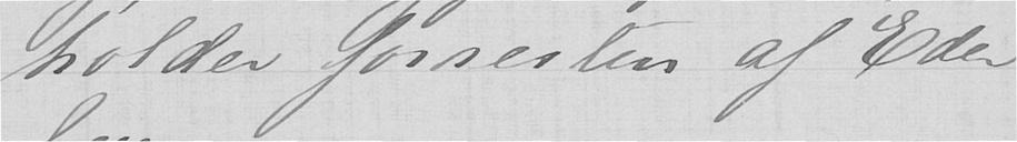holder forresten af Eder
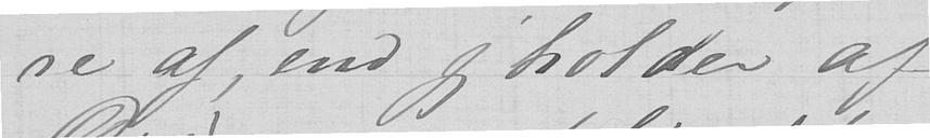re af end jeg holder af
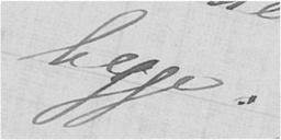begge.
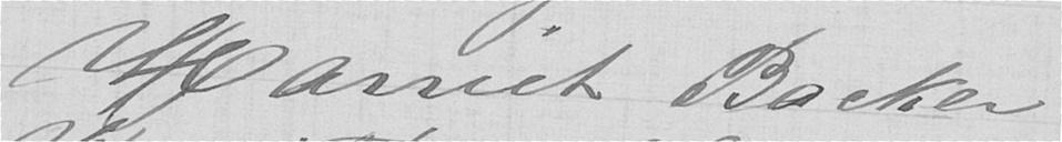Harriet Backer
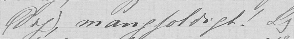Dig), mangfoldigt. Jeg
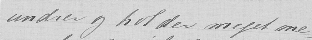undrer og holder meget me-
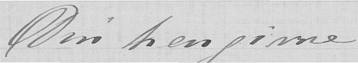Din hengivne
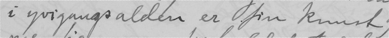i yvigangsalden er fin kunst,
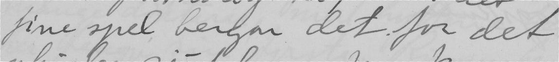fine spel bergar det for det
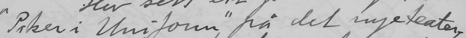"Piker i Uniform" på det nye teater
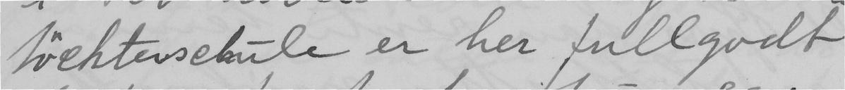töchterschule er her fullgodt
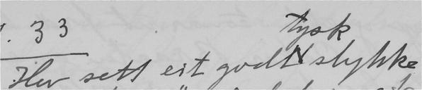Hev sett eit godt tysk stykke
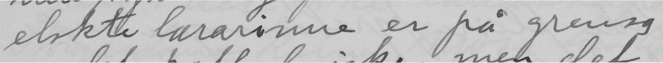elskte lærarinne er på grensa
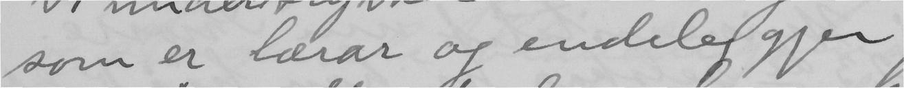som er lærar og endeleg gjer
